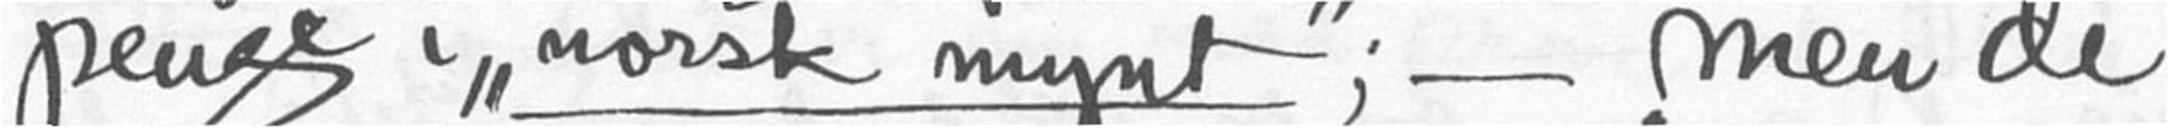penge i "norsk mynt"; - men de
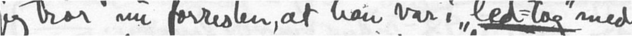jeg tror nu forresten, at han var i "led-tog" med
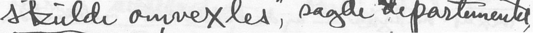"skulde omvexles" sagde departementet -
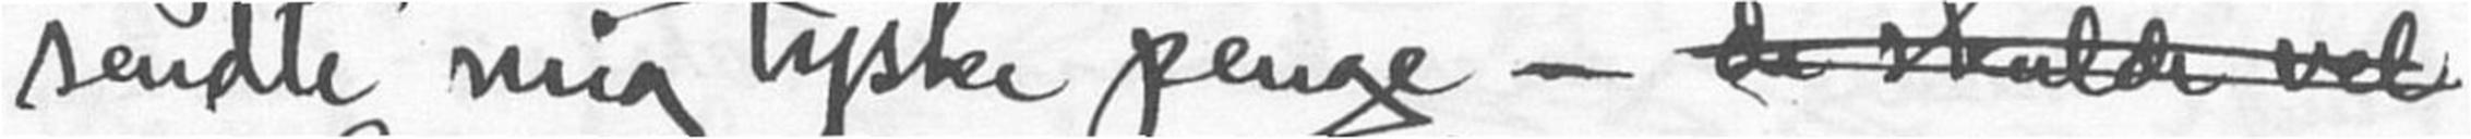sendte mig tyske penge - (de skulde vel
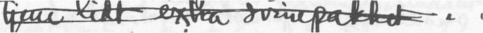tjene lidt extra svinepakket). -
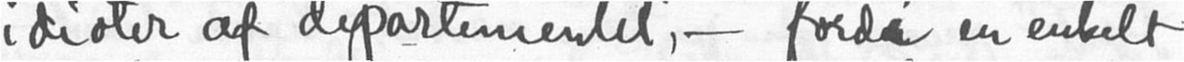idioter af departementet, - fordi en enkelt
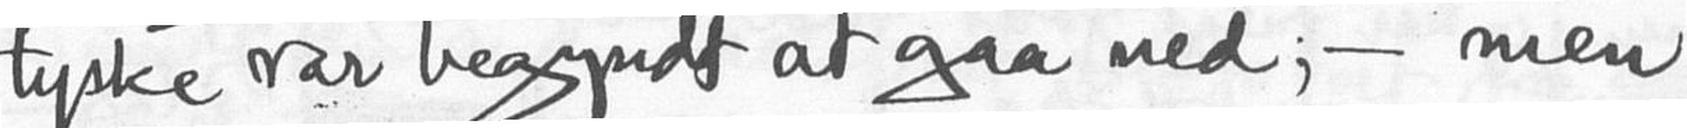tyske var begynt at gaa ned; - men
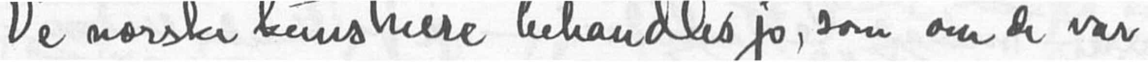De norske kunstnere behandles jo, som om de var
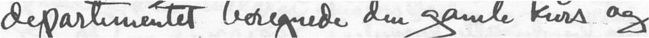departementet beregnede den gamle kurs og
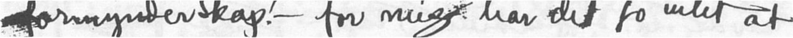formynderskap! - for mig har det jo intet at
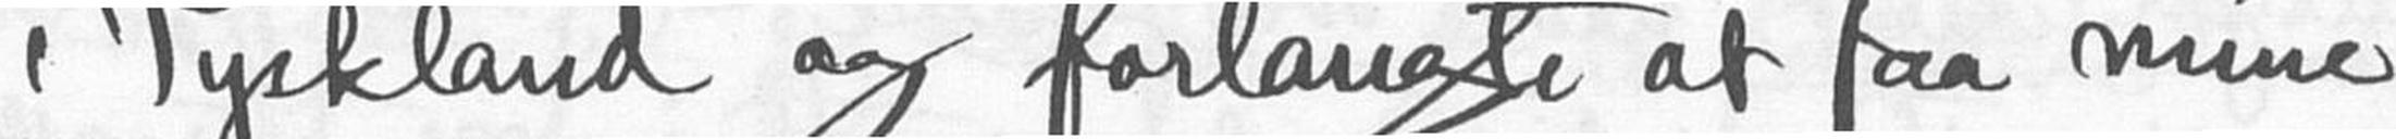i Tyskland og forlangte at faa mine
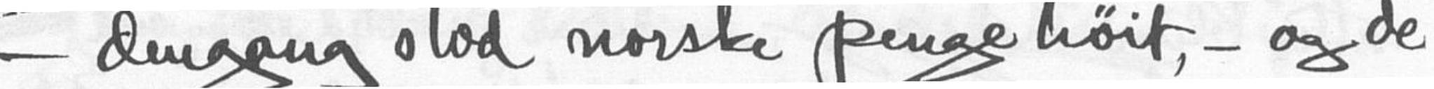dengang stod norske penge høit, - og de
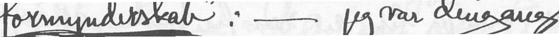"formynderskab": - jeg var dengang
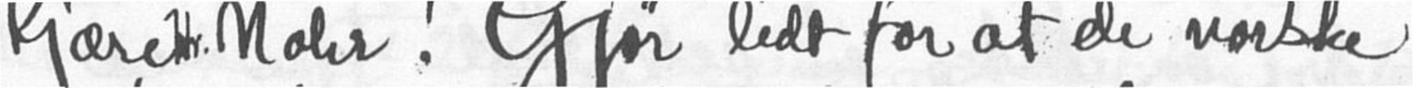Kjære Hr. Mohr! Gjør lidt for at de norske
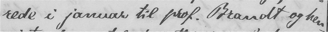rede i januar til prof. Brandt og hen-
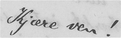Kjære ven!
